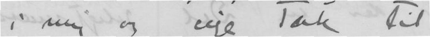i mig og sige Tak til
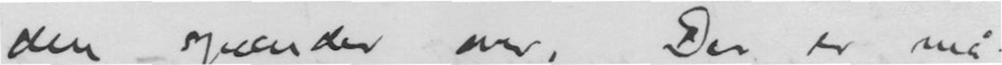den spænder over. Der er må-
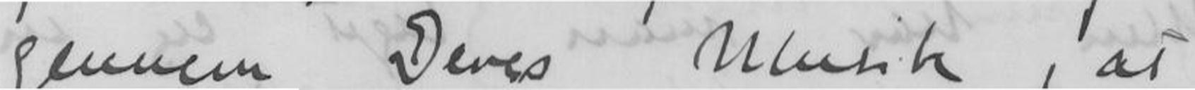gennem Deres Musik, at
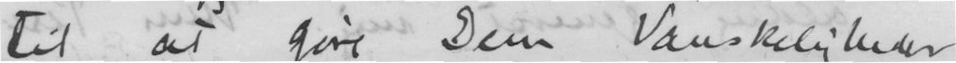til at gøre Dem Vanskeligheder
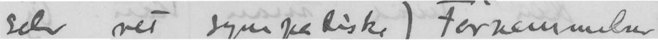selv ret sympatiske) Fornemmelser
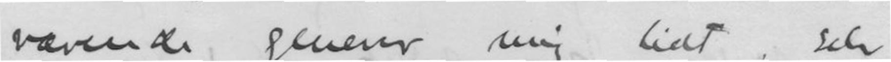værende generer mig lidt, selv
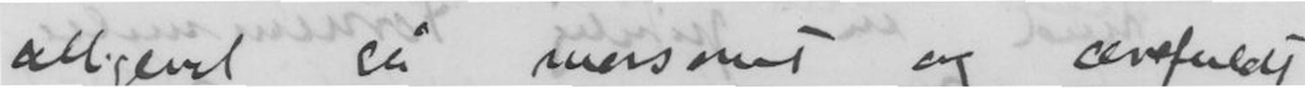alligevel så morsomt og ærefuldt
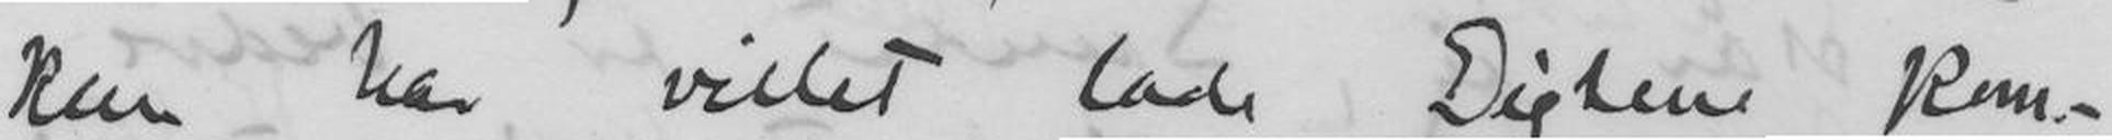kun har villet lade Digtene kom-
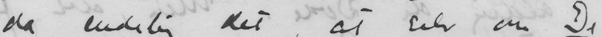da endelig det, at selv om De
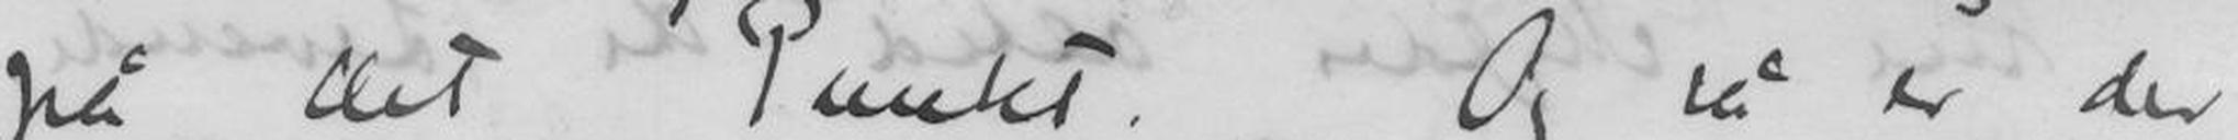på det Punkt. Og så er der
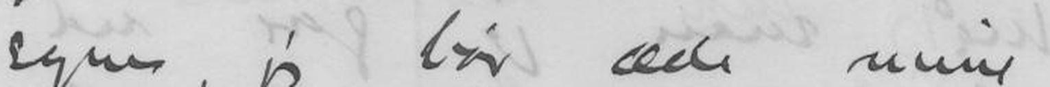syner, jeg bør dele mine
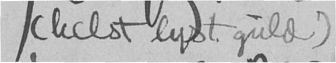(helst lyst guld)
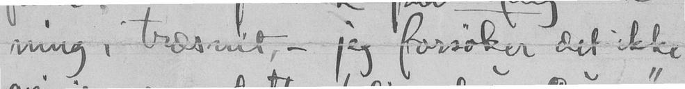ning i træsnit, - jeg forsøker det ikke
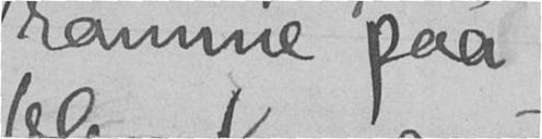ramme paa
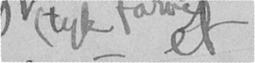(tyk farve)
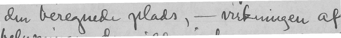den beregnede plads, - vurkningen af
